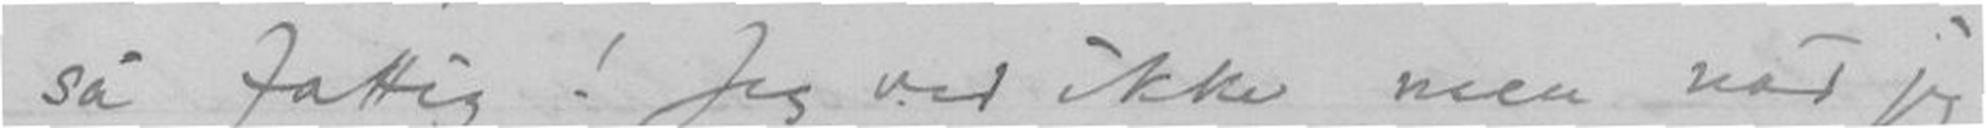så fattig! Jeg ved ikke mere når jeg
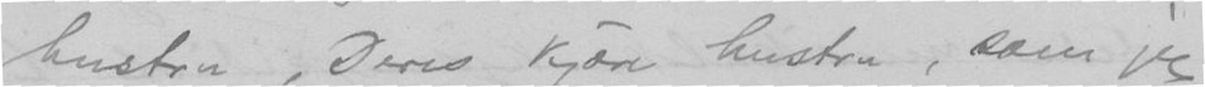hustru, Deres kjære hustru, som jeg
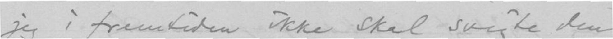jeg i fremtiden ikke skal svigte den
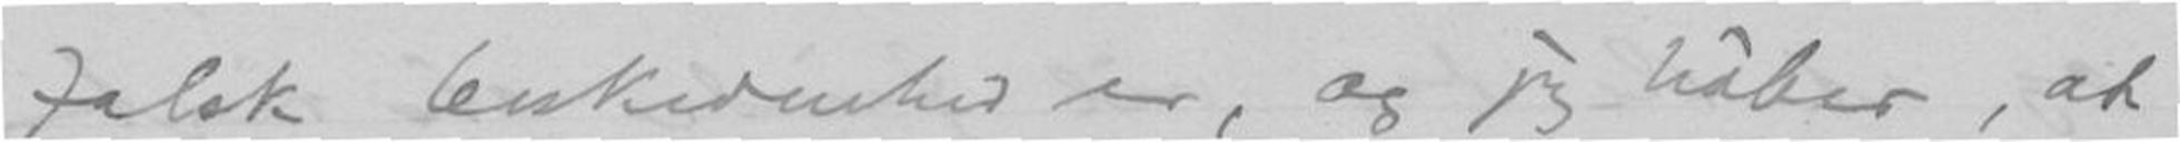falsk beskedenhed er, og jeg håber, at
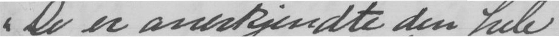"De er anerkjendt den hele
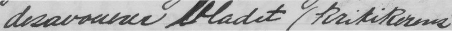desavouerer bladet (kritikerens)
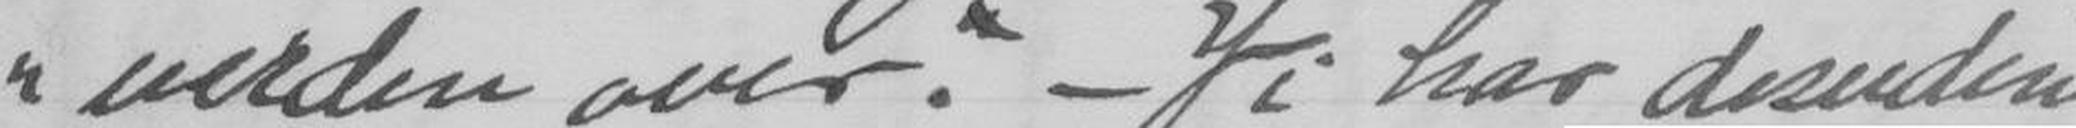"verden over." - Vi har desuden
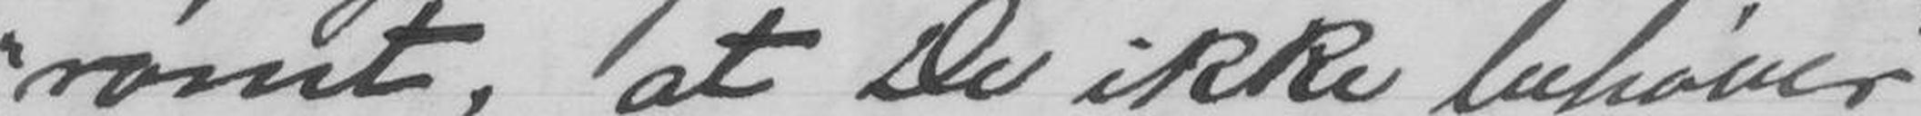"rømt, at De ikke behøver
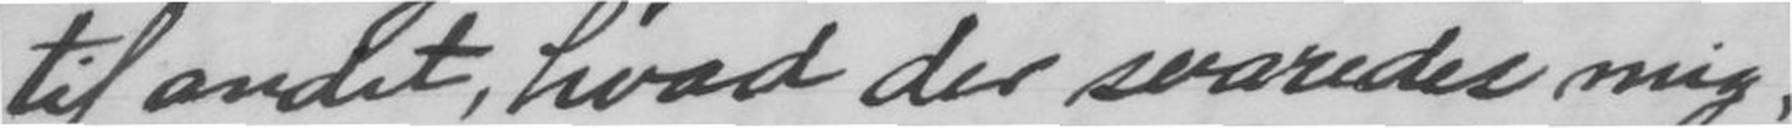til andet, hvad der svarede mig,
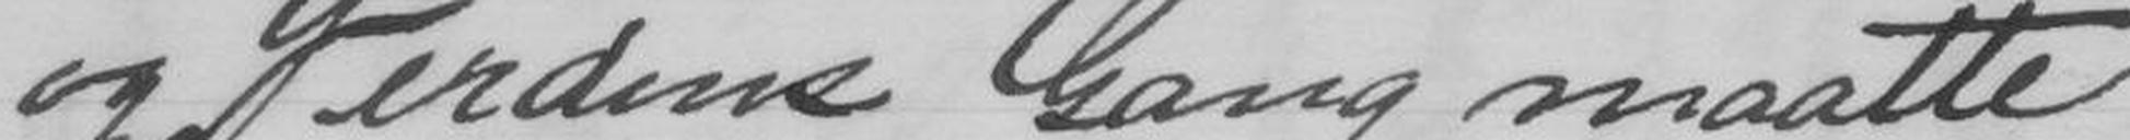og Verdens Gang maatte
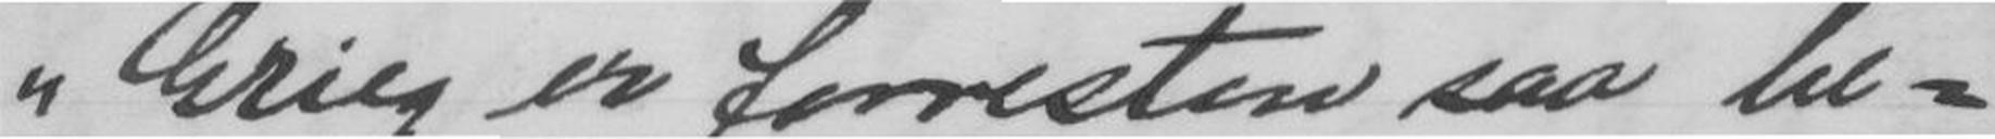"Grieg er forresten saa be-
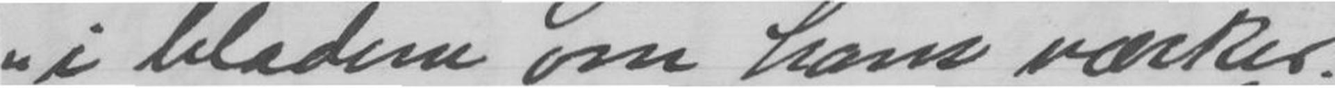"i bladern om hans værker
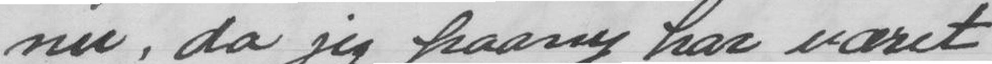nu, da jeg paany har været
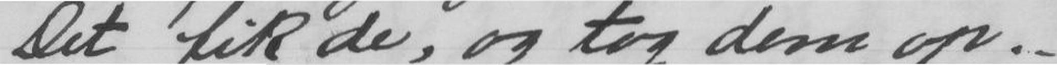Det fik de, og tog dem op. -
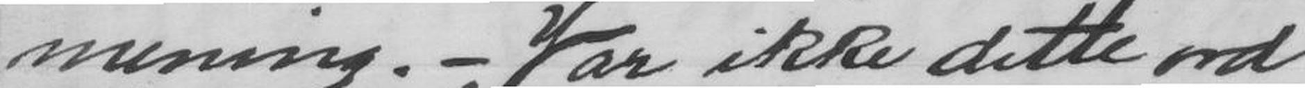mening. - Var ikke dette ord
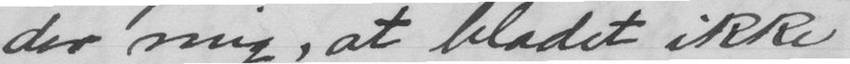der mig, at bladet ikke
